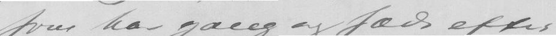som har gang og sæde efter
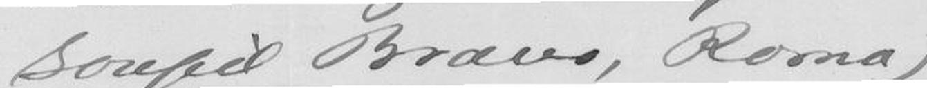konsul Bravo, Roma)
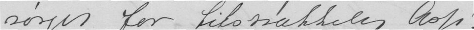sørget for tilstrækkelig Assi-
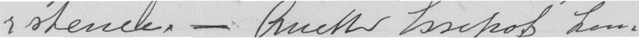stance. - Anette Essipof kom-
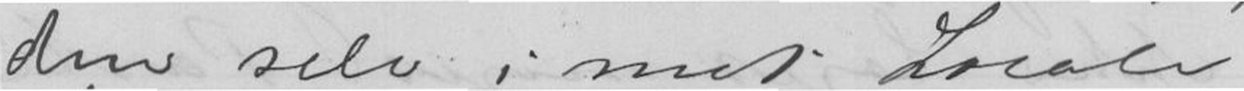den selv i mit Locale -
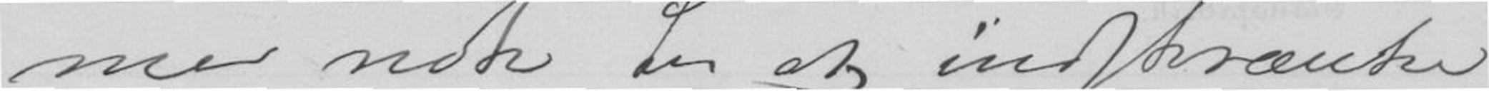=mer nok til at indskænke
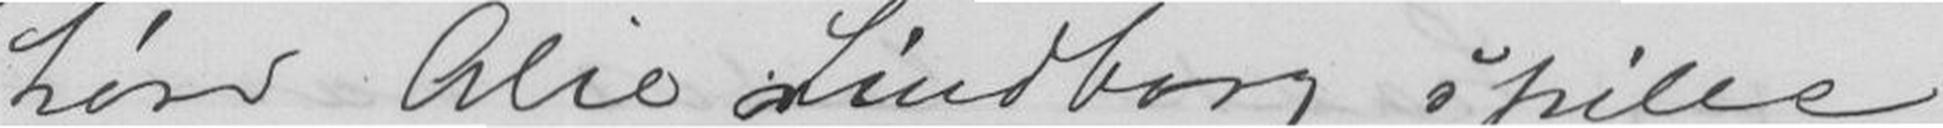høre Alie Lindberg spille
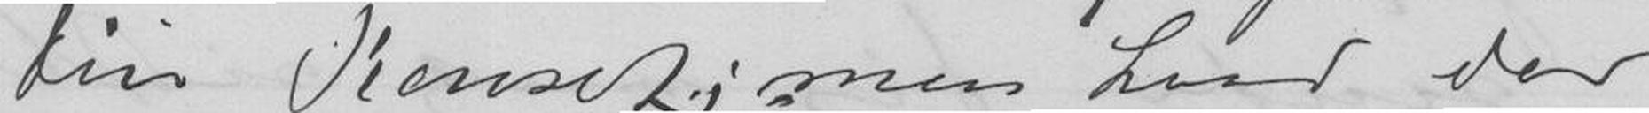Din Konsert; men hvad der
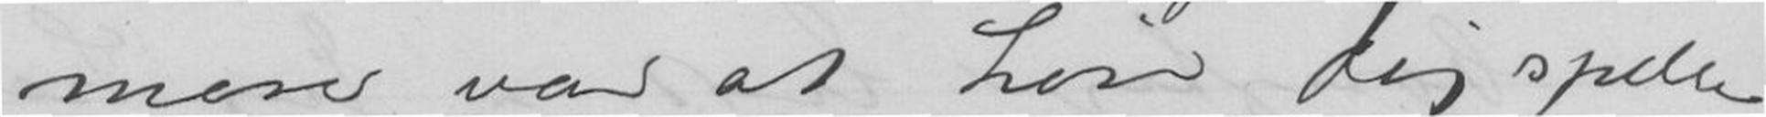mere var at høre Dig spille
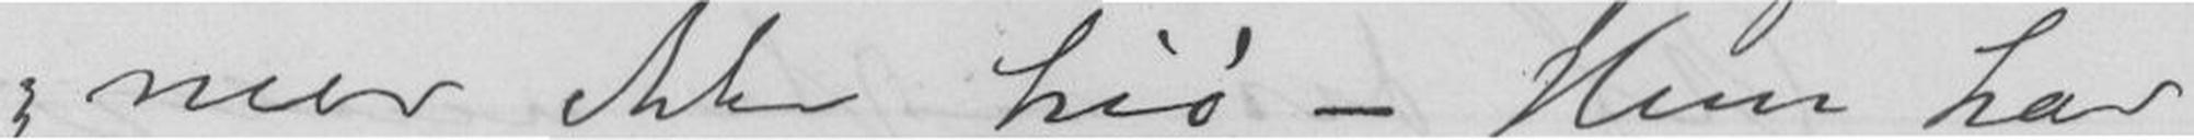mer ikke hid - Hun har
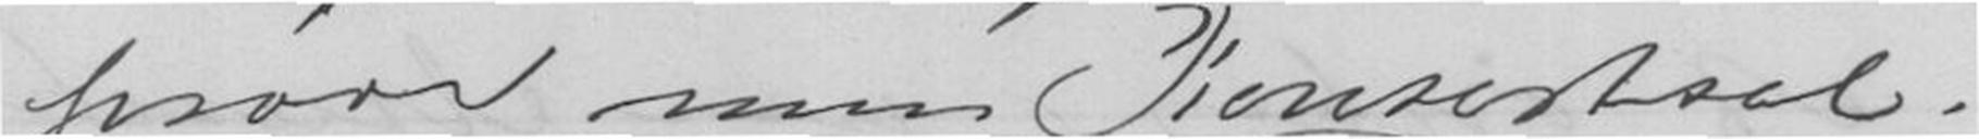prøve min Konsertsal.
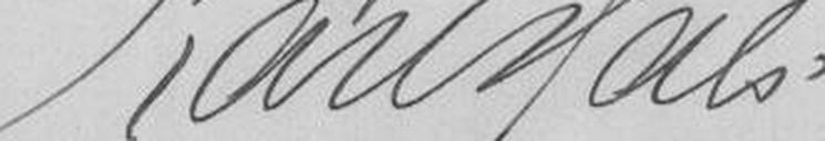Karl Hals
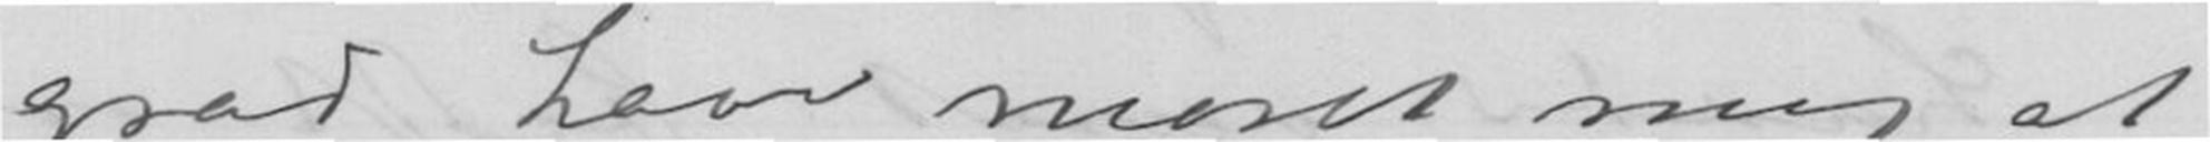grad have moret mig at
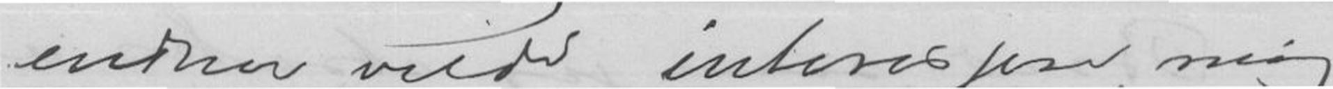endnu vilde interessere mig
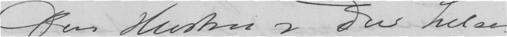Din Hustru og Du hilses
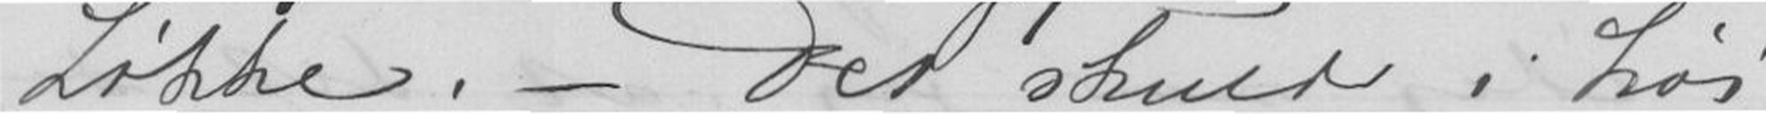Løkke. - Det skulde i høi
